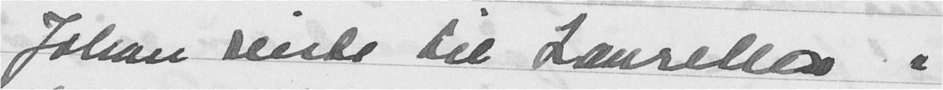Johan reiste til Lausellex i
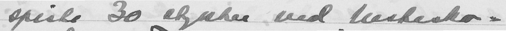spiste 30 stykker ved hestesko-
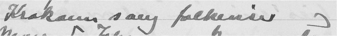Krokane sang folkeviser og
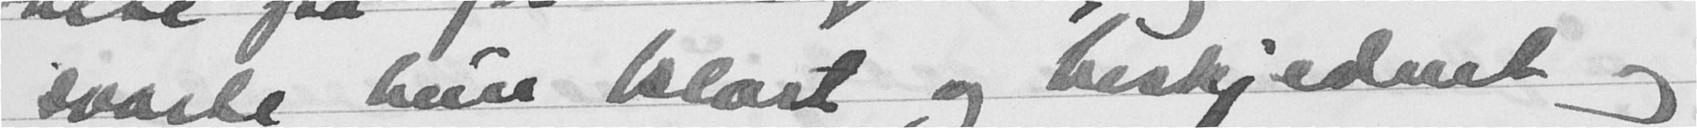svarte hun klart og beskjedent og
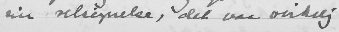sin velsignelse, det var virkelig
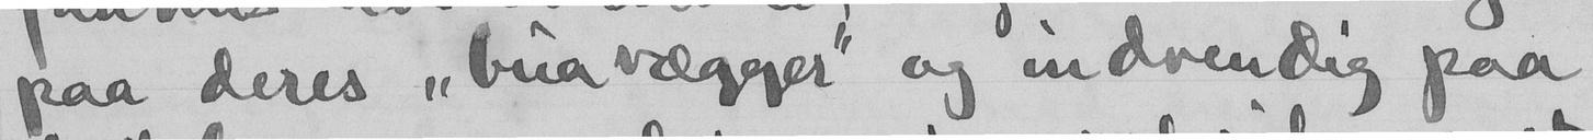paa deres "buavægger" og indvendig paa
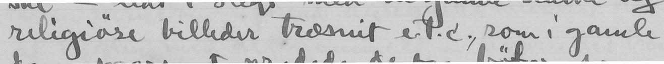religiøse billeder træsnit e.t.c., som i gamle
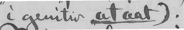i genitiv "ataat").
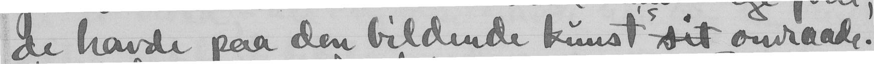de havde paa den bildende kunst  omraade.
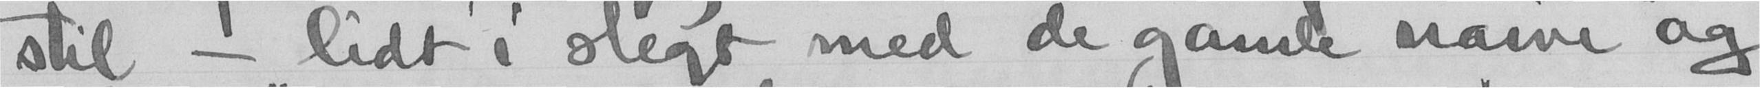stil - lidt i slegt med de gamle naive og
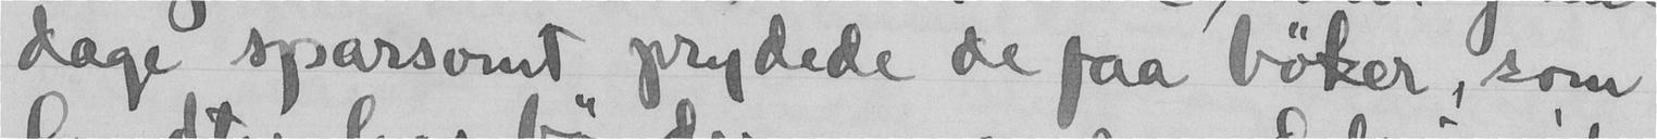dage sparsomt prydede de faa bøker, som
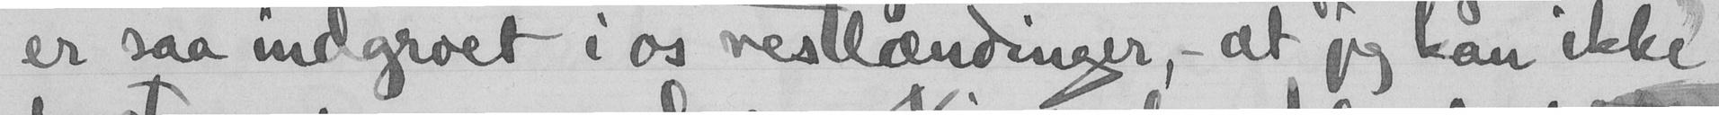er saa indgroet i os vestlændinger, - at jeg kan ikke
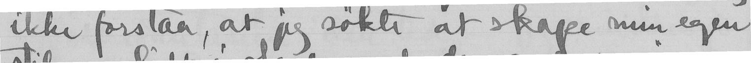ikke forstaa, at jeg søkte at skape min egen
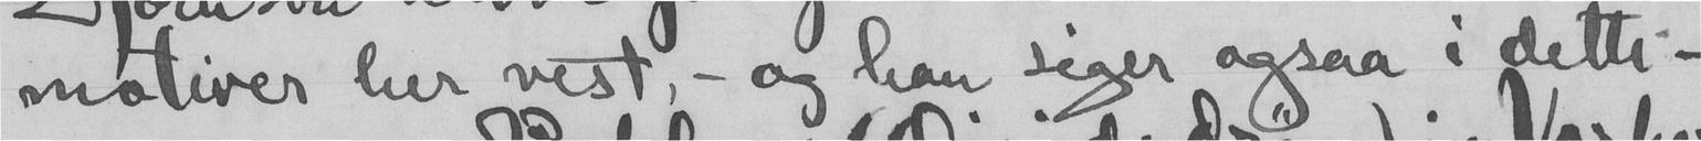motiver her vest, - og han siger ogsaa i dette-
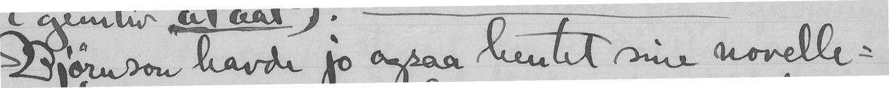Bjørnson havde jo ogsaa hentet sine novelle-
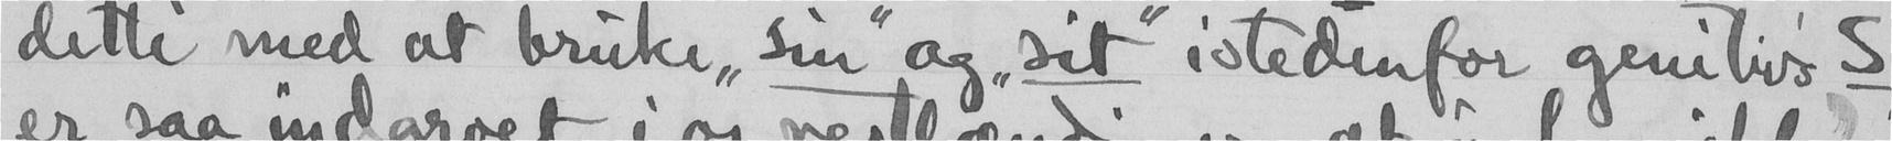dette med at bruke "sin" og "sit" istedenfor genitivs S
